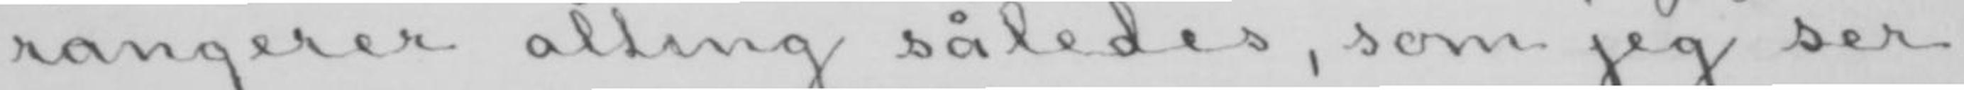rangerer alting således, som jeg ser
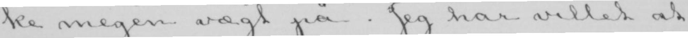ke megen vægt på. Jeg har villet at
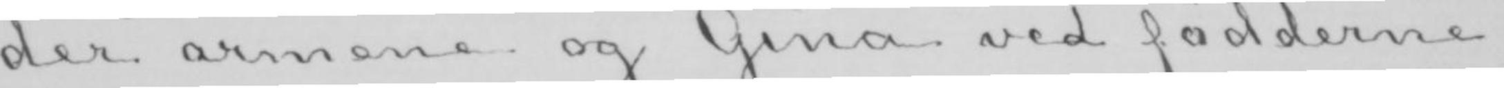der armene og Gina ved fødderne.
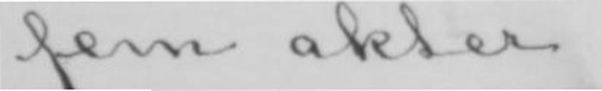fem akter.
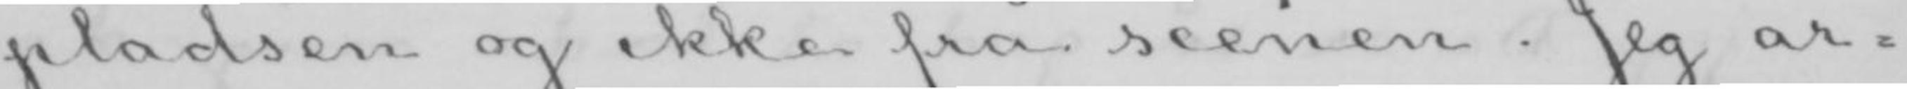pladsen og ikke fra scenen. Jeg ar-
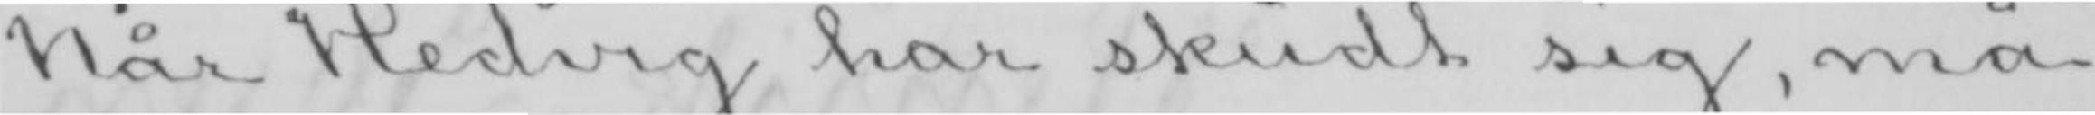Når Hedvig har skudt sig, må
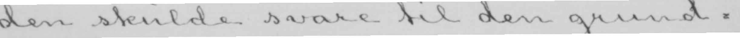den skulde svare til den grund-
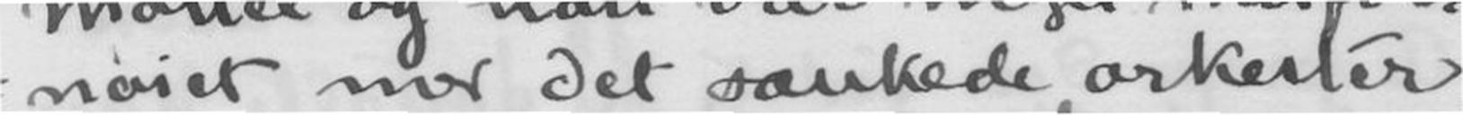nøiet med det sankede orkester.
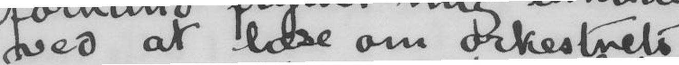ved at læse om orkestrets
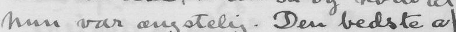hun var ængstelig. Den bedste af
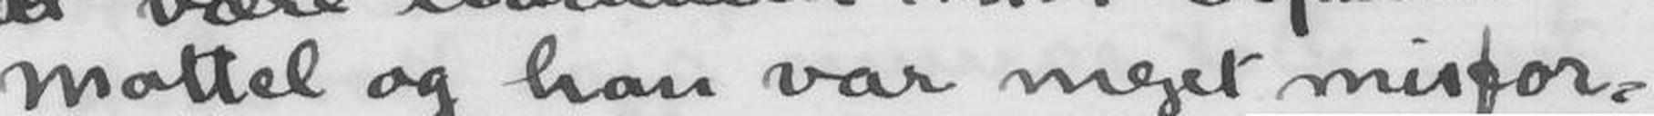Mottel og han var meget misfor-
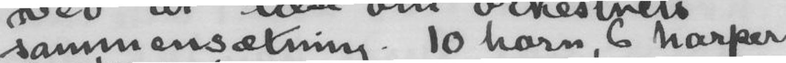sammensætning. 10 horn, 6 harper
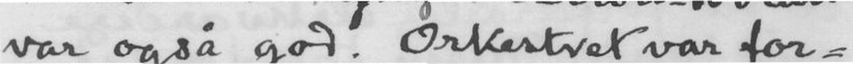var også god. Orkesteret var for-
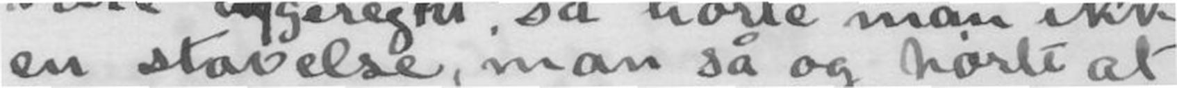en stavelse, man så og hørte at
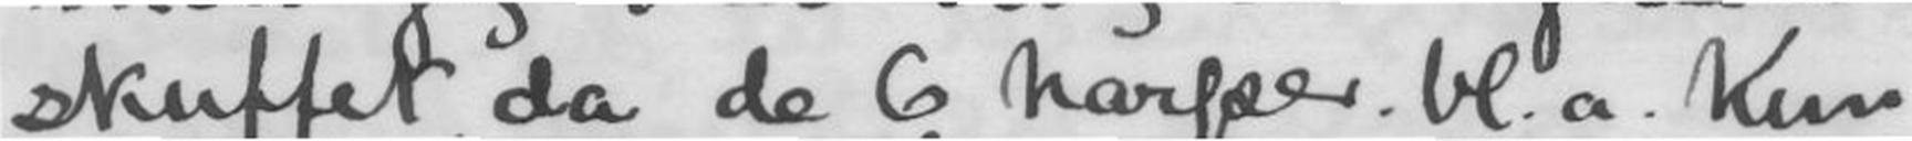skuffet, da de 6 harper bl.a. kun
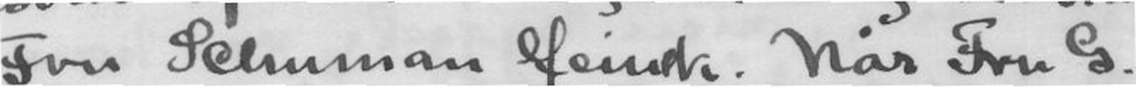Fru Schuman Heinck. Når Fru G.
